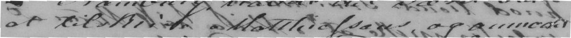at tilskrive Matthiassons, og anmodet
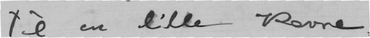til en lille korre-
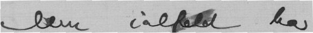Men ialfald har
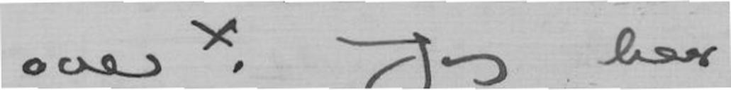over x. Jeg har
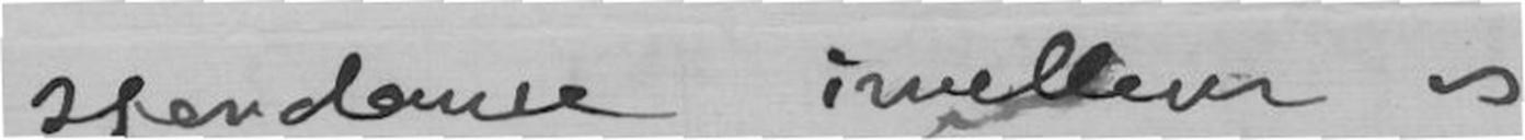spondance imellem os,
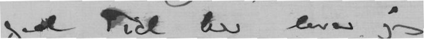god Tid her lever jeg
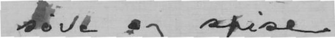sove og spise
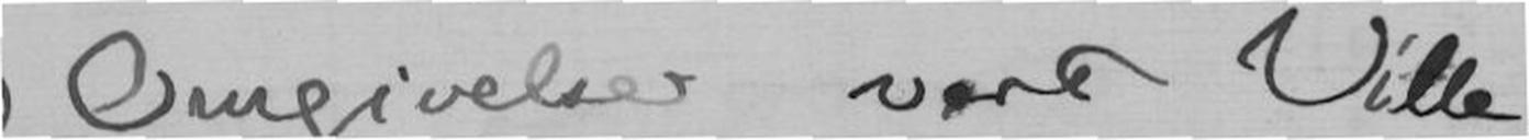Omgivelser vort Villa
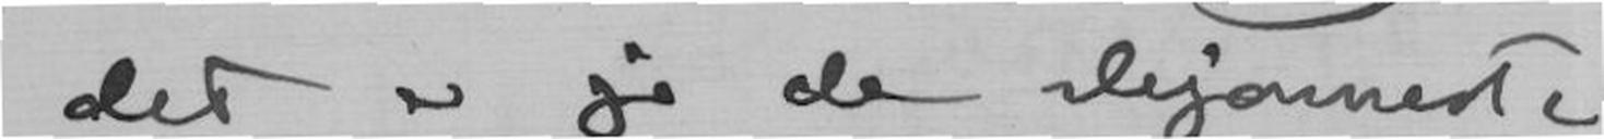det er jo de skjønneste
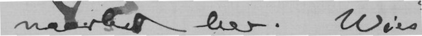mærket her. Wies
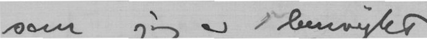som jeg er henrykt
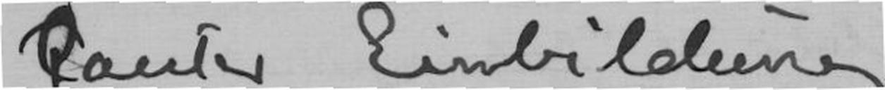Fanter Einbildung -
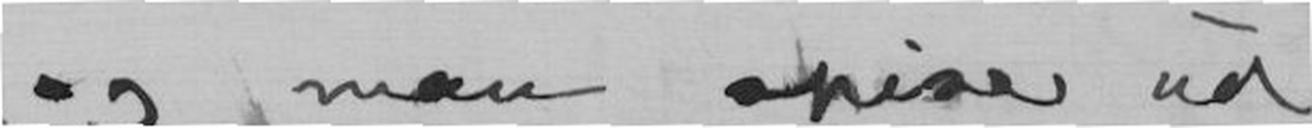og man spiser ud
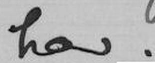har.
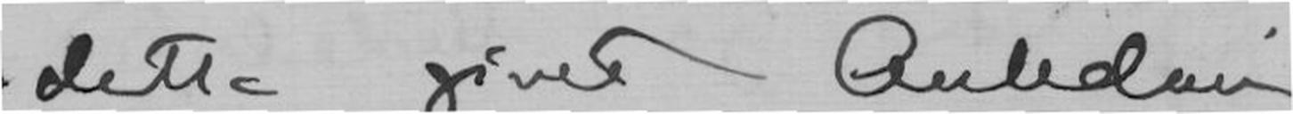dette givet Anledning
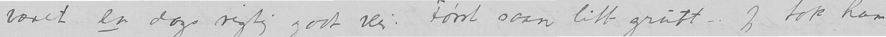været en dags rigtig godt veir. Først saan litt grått –. Jeg tok ham
